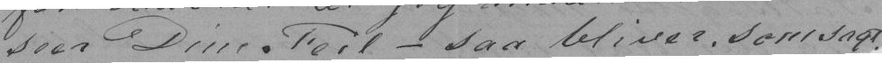seer Dine Feil - saa bliver, som sagt
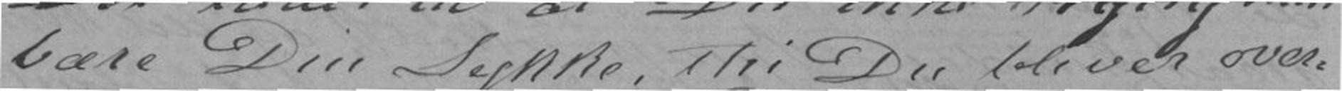bære Din Lykke, thi Du bliver over-
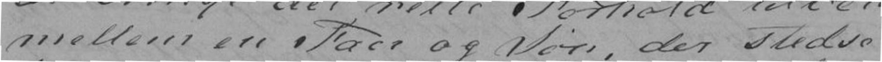mellem en Faer og Søn, der stedse
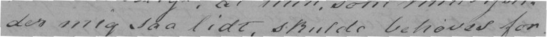der mig saa lidt, skulde behøves for
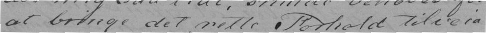at bringe det rette Forhold tilveie
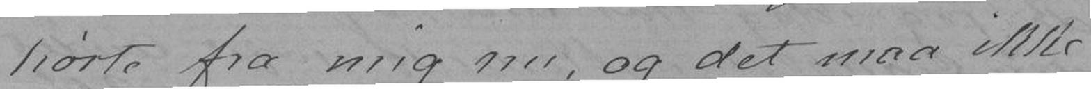hørte fra mig nu, og det maa ikke
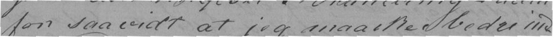for saavidt at jeg maaske bedre ind-
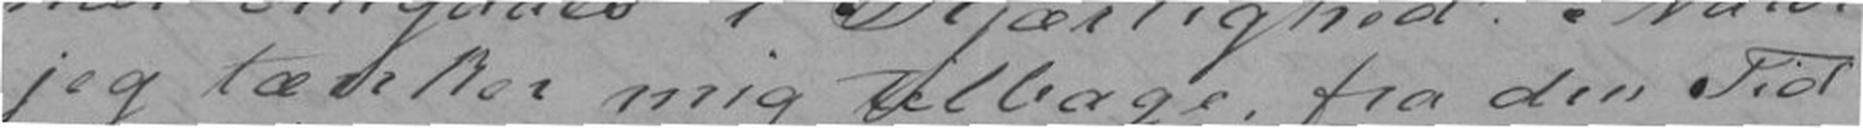jeg tænker mig tilbage, fra den Tid
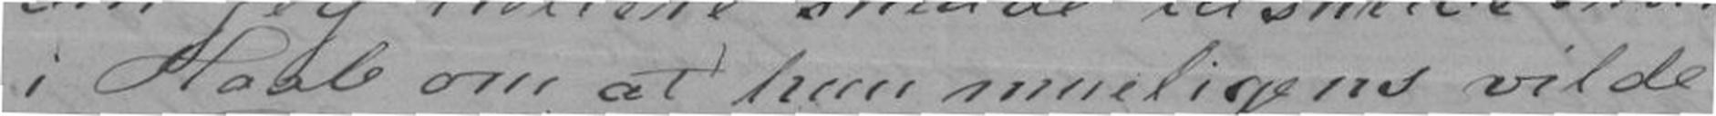i Haab om at hun mueligens vilde
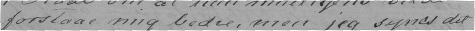forstaae mig bedre, men jeg synes det
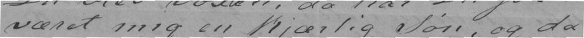været mig en kjærlig Søn, og da
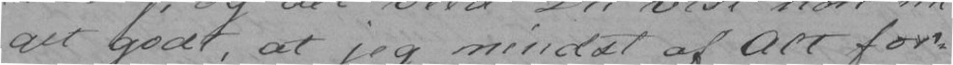get godt, at jeg mindst af Alt for-
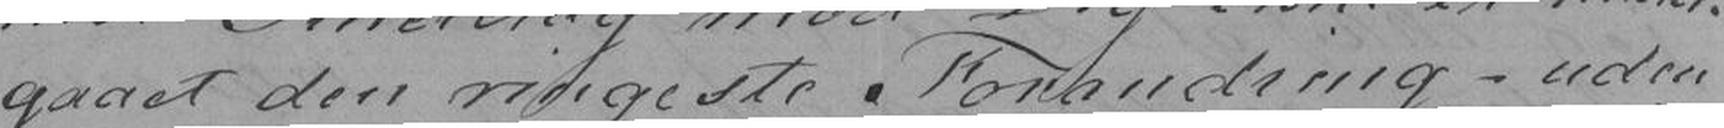gaaet den ringeste Forandring - uden
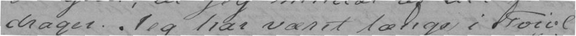drager. Jeg har været længe i Tvivl
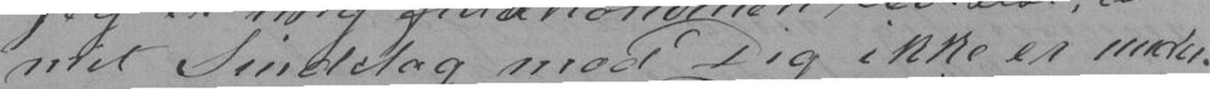mit Sindelag mod Dig ikke er under-
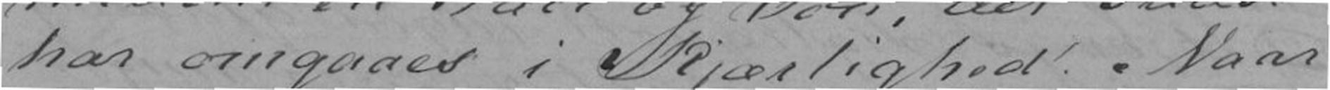har omgaaes i Kjærlighed. Naar
